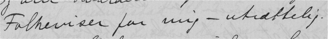Folkvriser for mig - utrættelig.
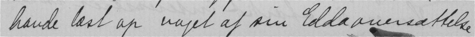havde læst op noget af sin Eddaoversættelse
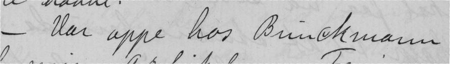- Var oppe hos Brinckmann
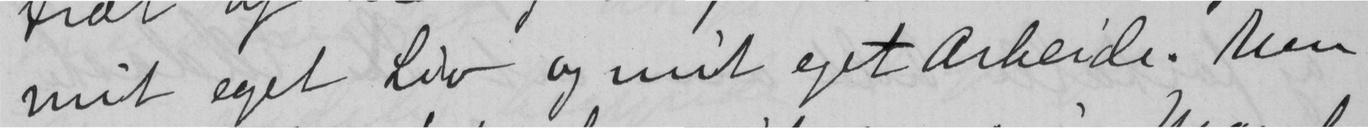mit eget Liv og mit eget arbeide. Men
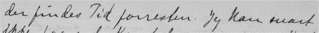der findes Tid forresten. Jeg kan snart
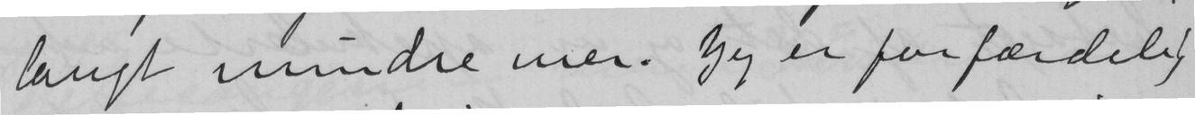langt mindre mer. Jeg er forfærdelig
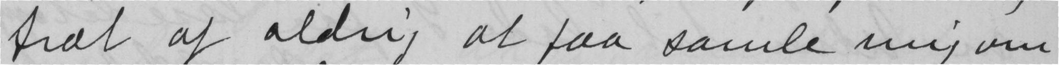træt af aldrig at faa samle mig om
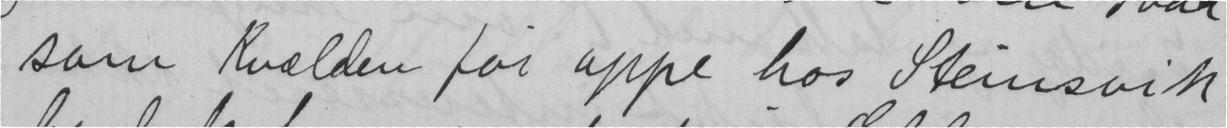som Kvælden for oppe hos Steinsvik
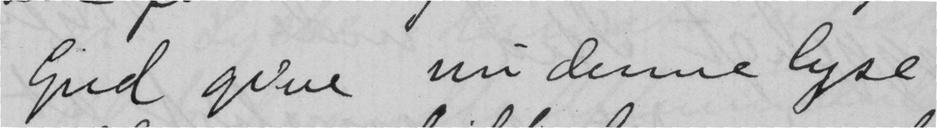Gud give nu denne lyse
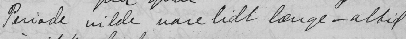Periode vilde vare lidt længe - altid
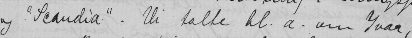og "Scandia". Vi talte bl. a. om Ivar,
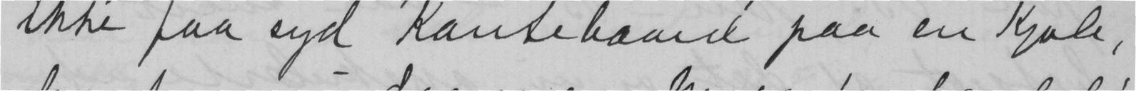ikke faa syd Kantebaand paa en Kjole,
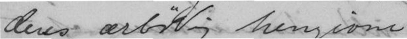deres ærbødig hengivne
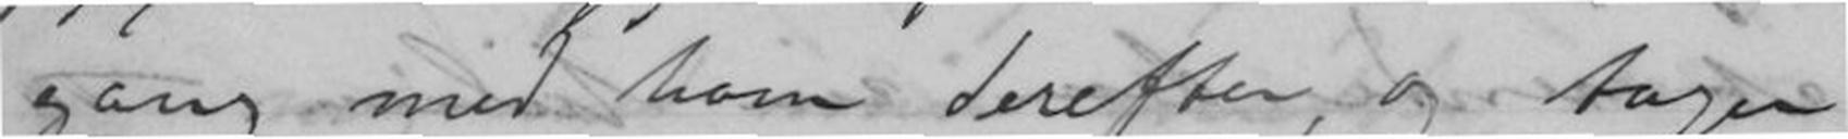gang med ham derefter, og tager
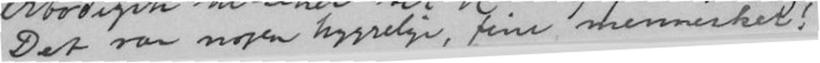Det var nogen hyggelige, fine mennesker!
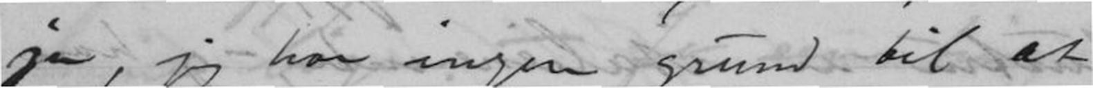ja, jeg har ingen grund til at
